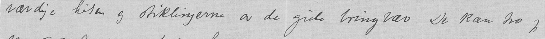værdige hilsen og stiklingerne av de gule bringbær. De kan tro jeg
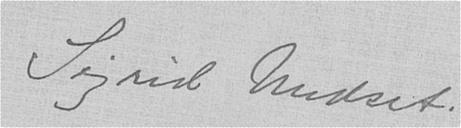Sigrid Undset.
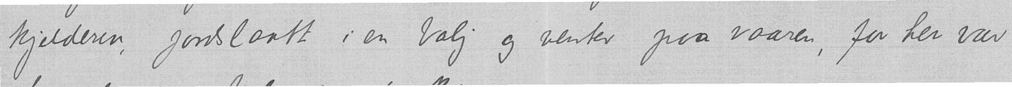kjelderen, jordslaatt i en balj og venter paa vaaren, for her var
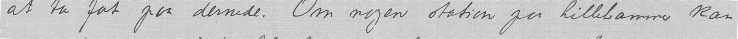at ta fat paa dernede. Om nogen station paa Lillehammer kan
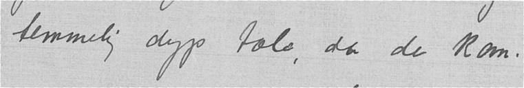temmelig dyp tæle, da de kom.
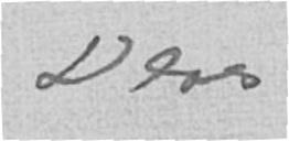Deres
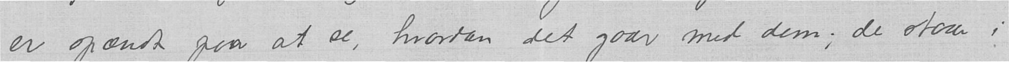er spændt paa at se, hvordan det gaar med dem; de staar i
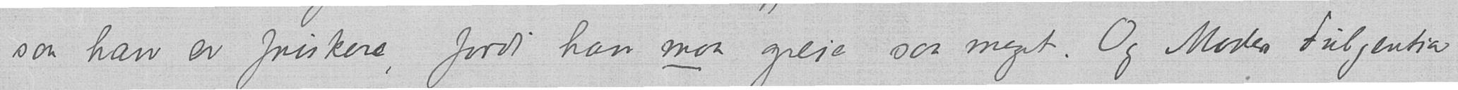saa han er friskere, fordi han maa greie saa meget. Og Moder Fulgentia
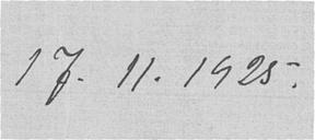17.11.1925.
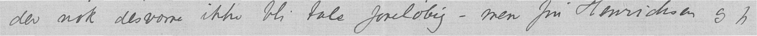der nok desværre ikke bli tale foreløbig – men fru Henrichsen og jeg
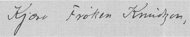Kjære Frøken Knudzon,
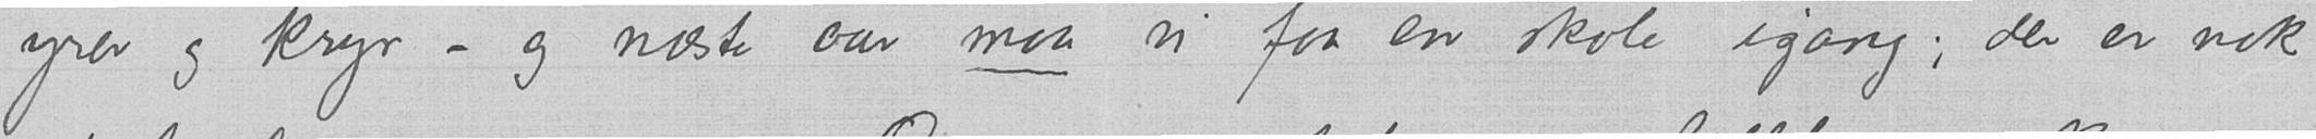yrer og kryr – og næste aar maa vi faa en skole igang; der er nok
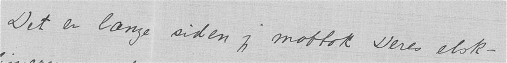Det er længe siden jeg mottok Deres elsk-
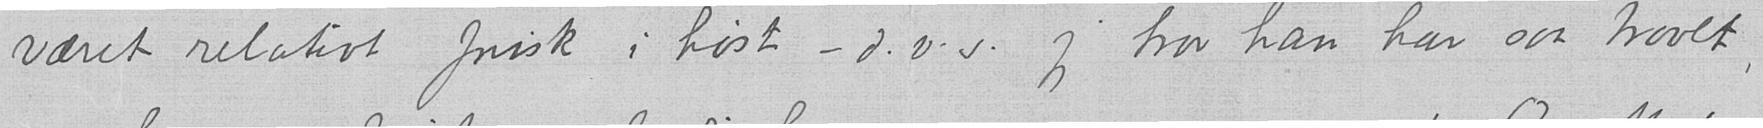været relativt frisk i høst – d.v.s. jeg tror han har saa travlt,
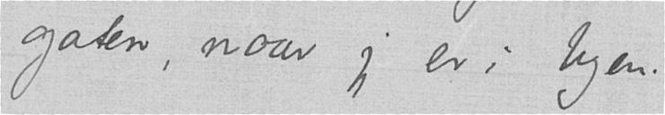gaten, naar jeg er i byen.
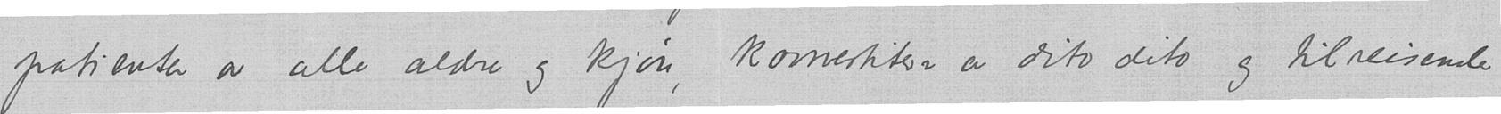patienter av alle aldre og kjøn, konvertiter av dito dito og tilreisende
